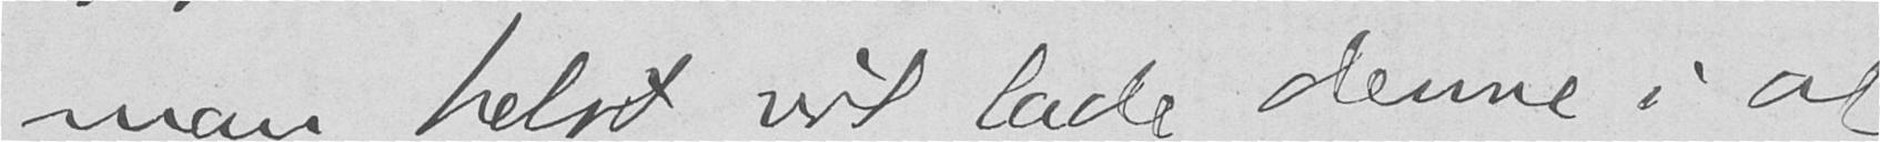man helst vil lade denne i al
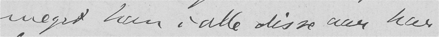meget han i alle disse aar har
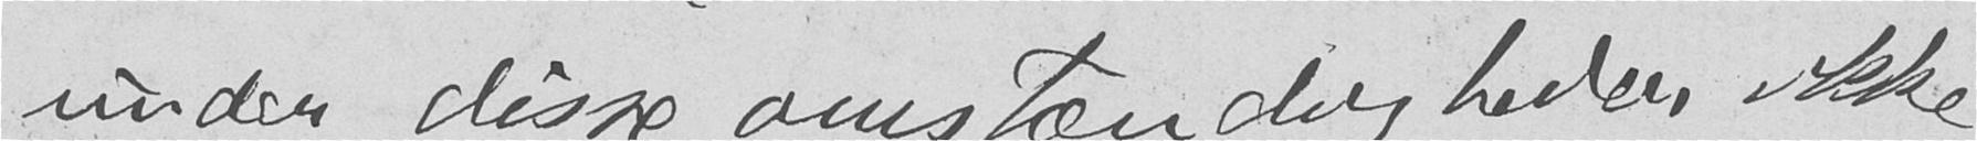under disse omstændigheder ikke,
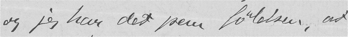og jeg har det paa følelsen, at
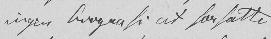ingen biografi at forfatte.
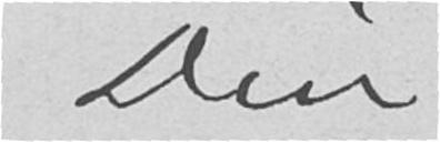Din
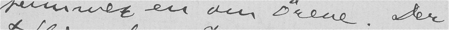summer en om ørene. Der
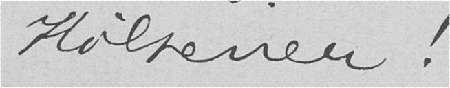Hilsener!
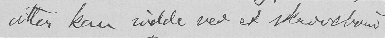atter kan sidde ved et skrivebord
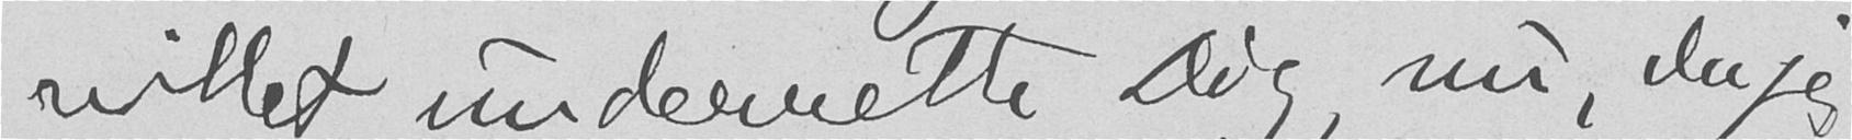villet underrette Dig, nu, da jeg
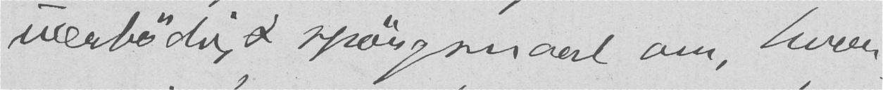uærbødigt spørgsmaal om, hvor-
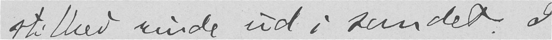stilhed rinde ud i sandet. I
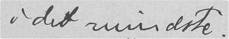i det mindste.
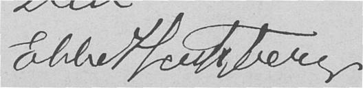Ebbe Hertzberg.
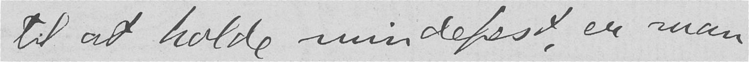til at holde mindefest, er man
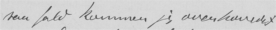saa fald kommer jeg overhovedet
